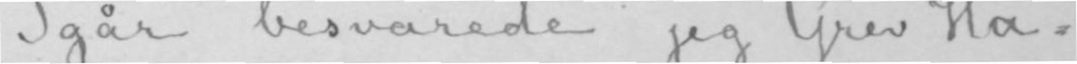Igår besvarede jeg Grev Ha-
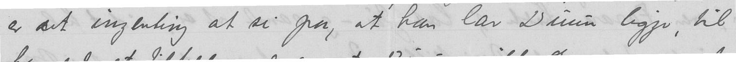er det ingenting at si paa, at han lar Duun ligge, til
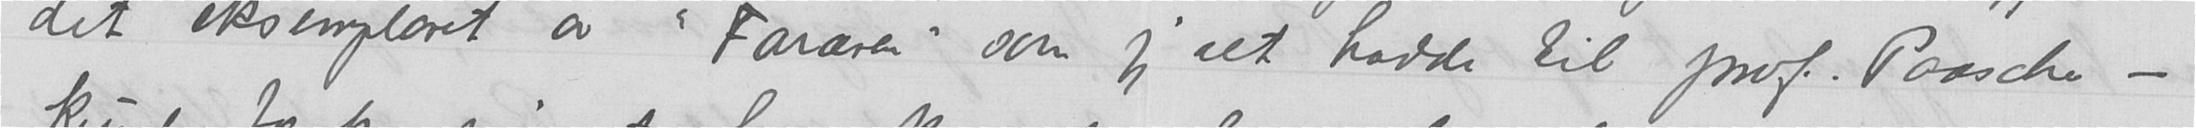det eksemplaret av "Fararen" som jeg alt hadde til prof. Paasche -
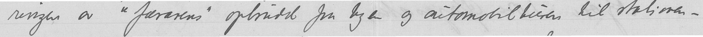ringen av "fararens" opbrudd fra byen og automobilturen til stationen –
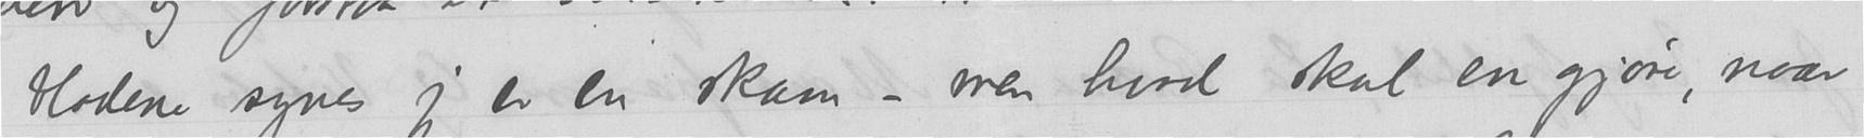bladene synes jeg er en skam – men hvad skal en gjøre, naar
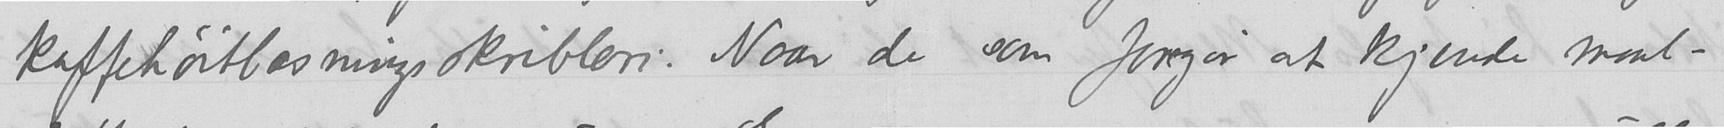kaffehøitlasningsskribleri. Naar de som foregir at kjende maal-
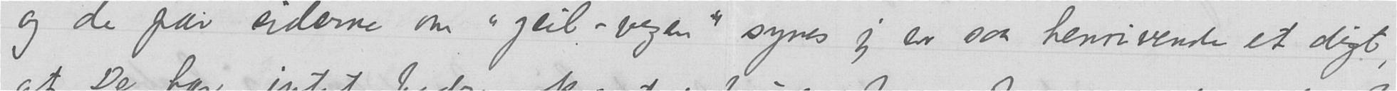og de par siderne om "geil-vegen" synes jeg er saa henrivende et digt,
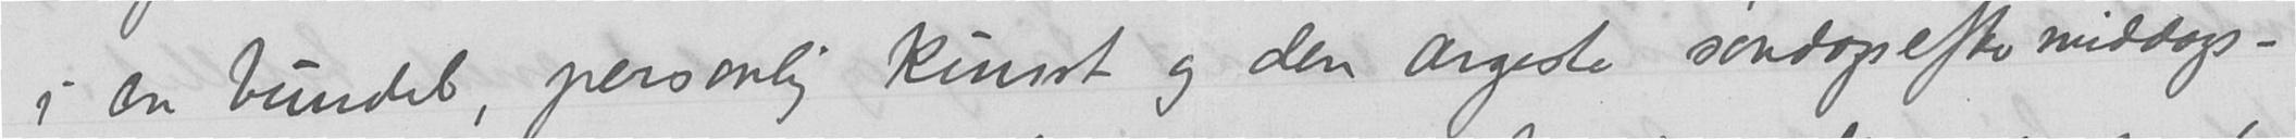i en bundet, personlig kunst og den argeste søndagseftermiddags-
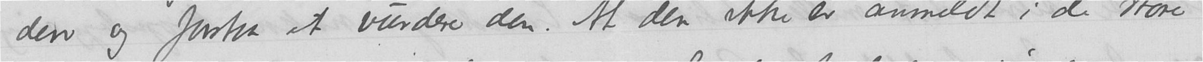den og forstaa at vurdere den. At den ikke er anmeldt i de store
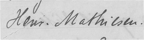Henr. Mathiesen.
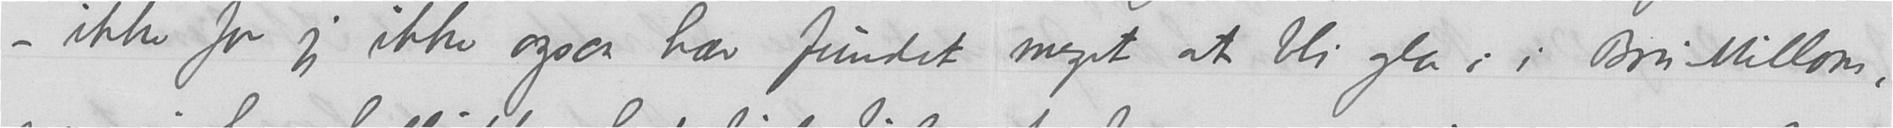– ikke for jeg ikke ogsaa har fundet meget at bli gla i i Bru-Millom,
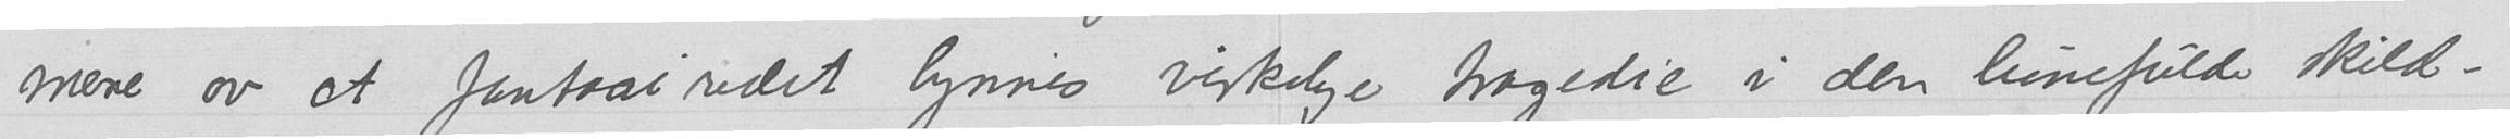mere av et fantasiredet lynnes virkelige tragedie i den lunefulde skild-
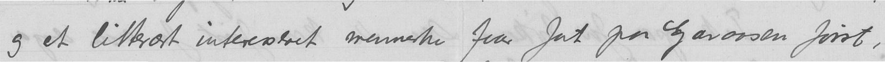og et litterært interesseret menneske faar fat paa Garaasen først,
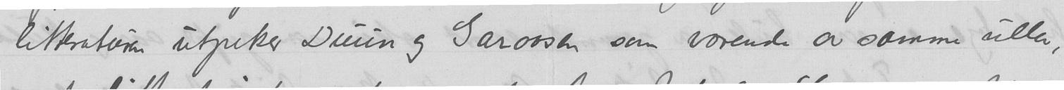litteraturen utpeker Duun og Garaasen som værende av samme ullen,
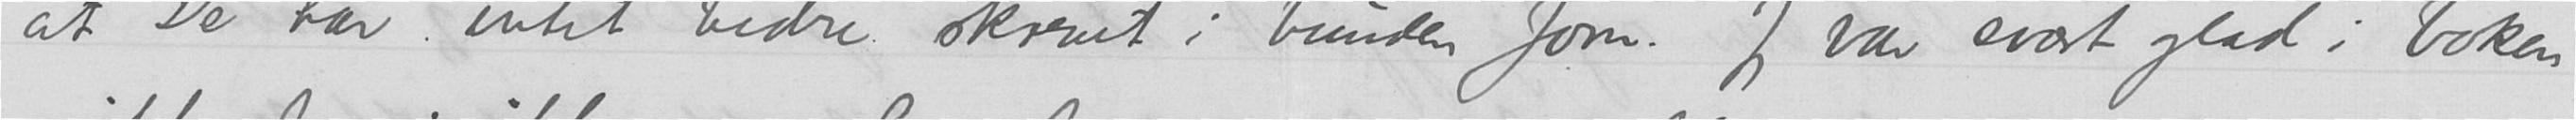at De har intet bedre skrevet i bunden form. Jeg var svært glad i boken
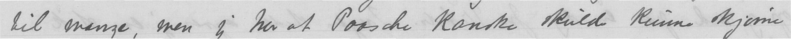til mange, men jeg tror at Paasche kanske skulde kunne skjønne
